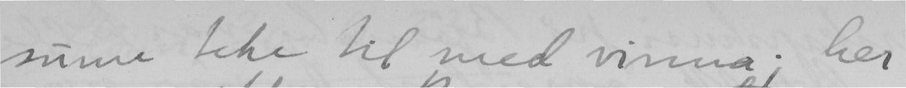sume teke til med vinna; her
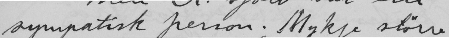sympatisk person. Mykje större
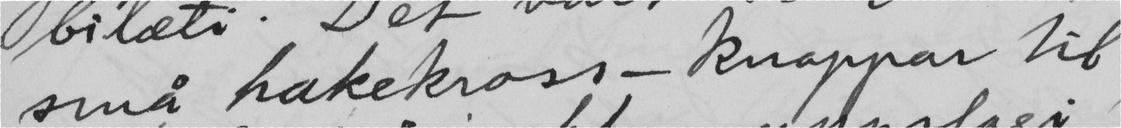små hakekross-knappar til
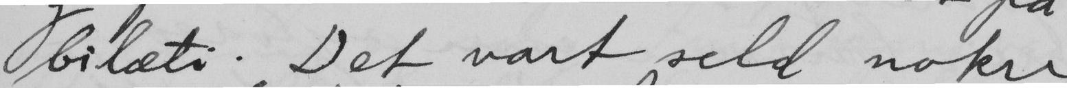bilæti. Det vart seld nokre
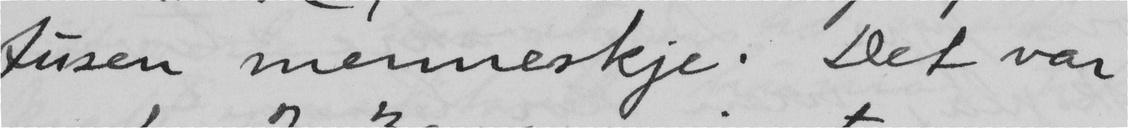tusen menneskje. Det var
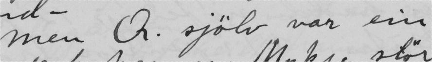Men Q. sjölv var ein
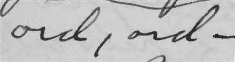ord, ord –
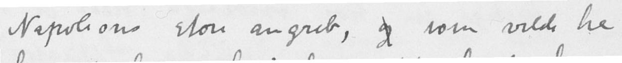Napoleons store angreb, og som vilde ha
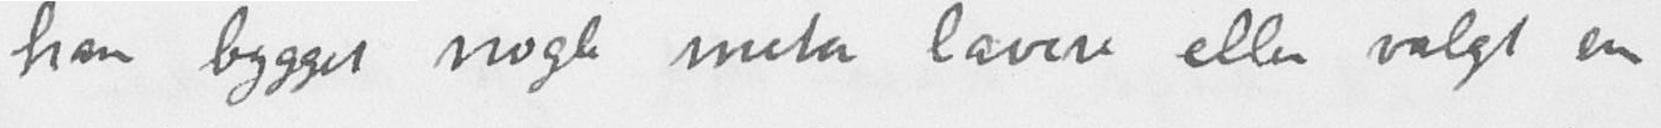han bygget nogle meter lavere eller valgt en
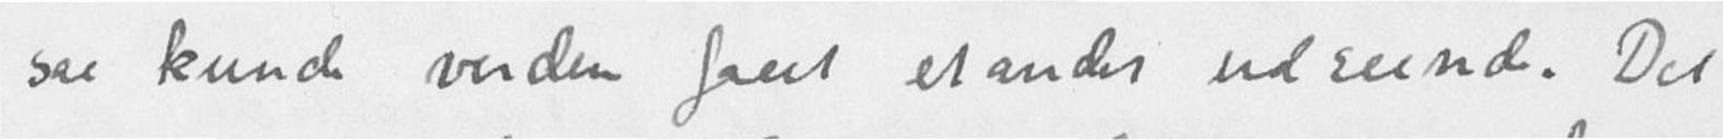saa kunde verden faaet et andet udseende. Det
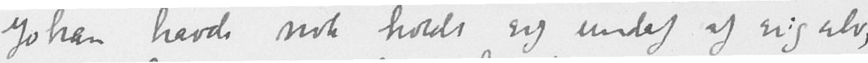Johan havde nok holdt sig undaf af sig selv,
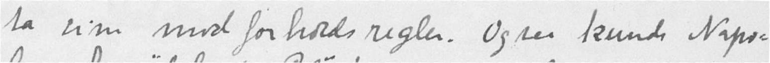ta sine modforholdsregler. Ogsaa kunde Napo-
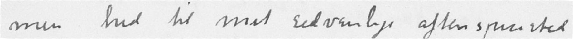men hid til mit sedvanlige aftenspisested
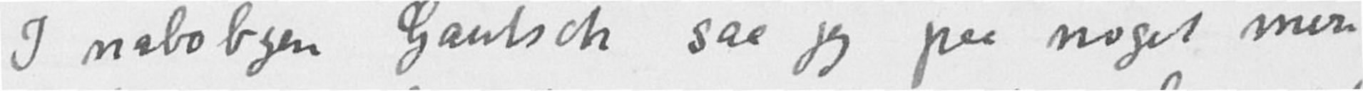I nabobyen Gautsch saa jeg paa noget mere
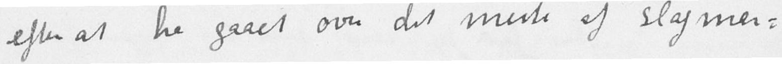efter at ha gaaet over det meste af slagmar-
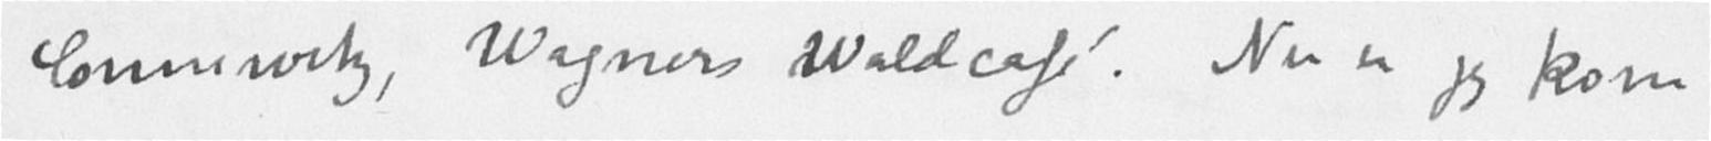Connewitz, Wagners Waldcafé. Nu er jeg kom-
